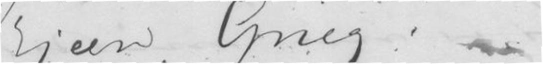Kjære Grieg!
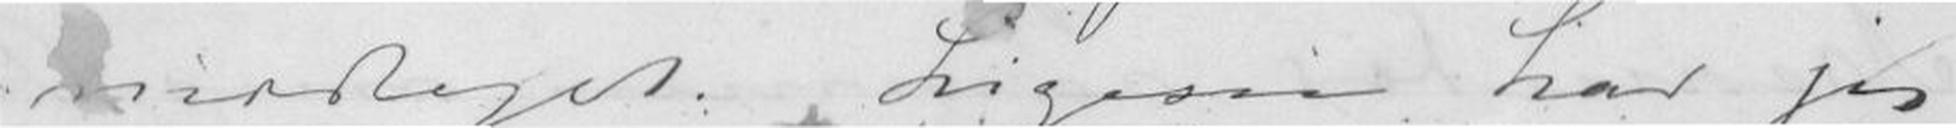modtaget. Ligesom har jeg
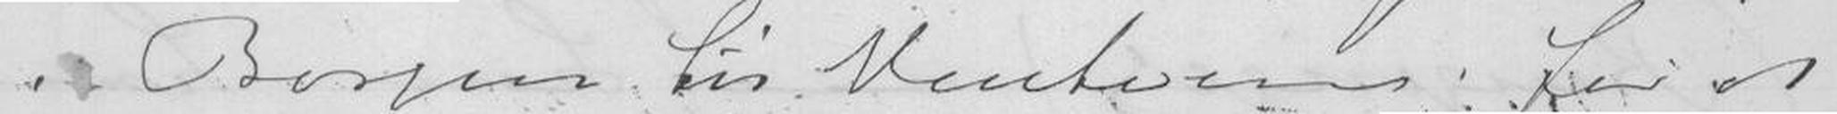i Bergen til Vinteren for at
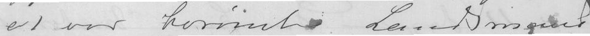at vor berømte Landsmand
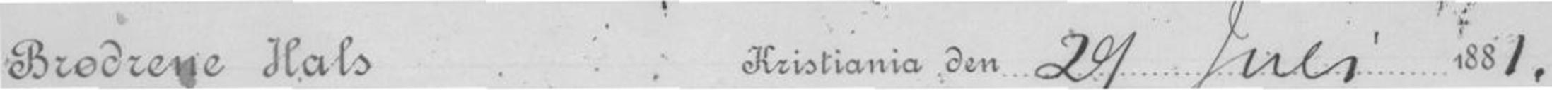Brødrene Hals Kristiania den 29 Juli 1881.
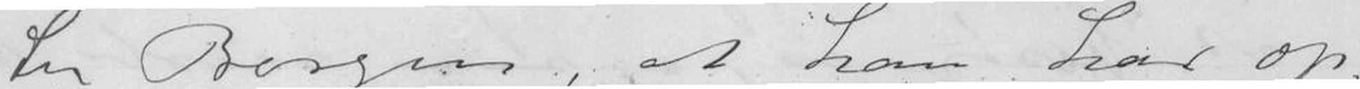til Bergen, at han har op-
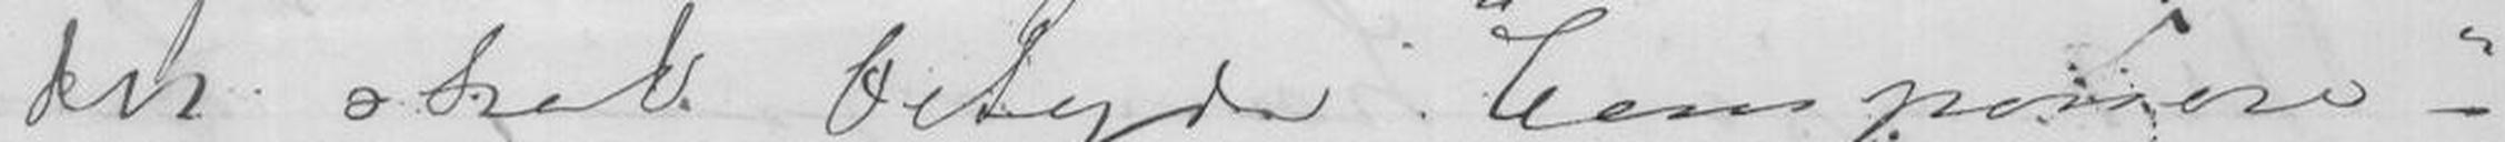det skal betyde "Componere"-
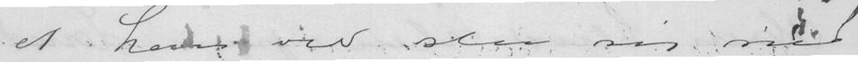at hans vil slaa sig ned
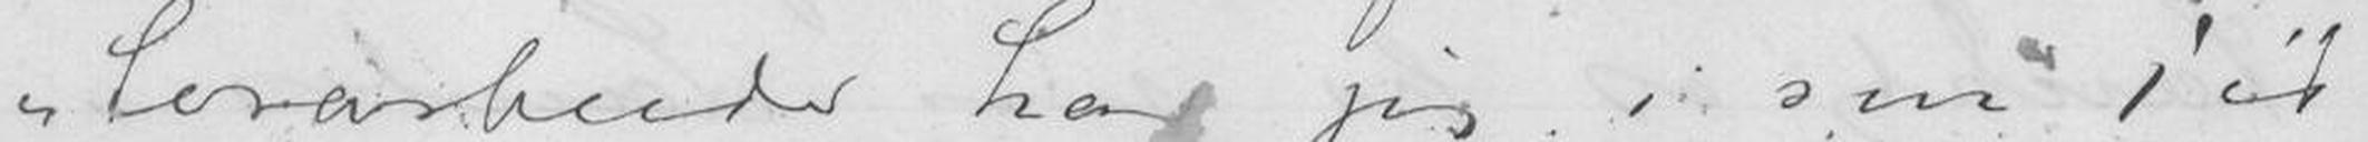terarbeide har jeg i sin Tid
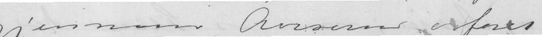gjennem Aviserne erfaret
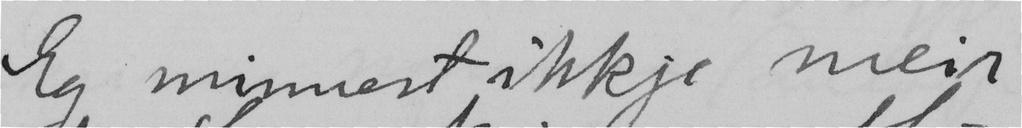Eg minnest ikkje meir
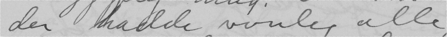der hadde vonleg alle
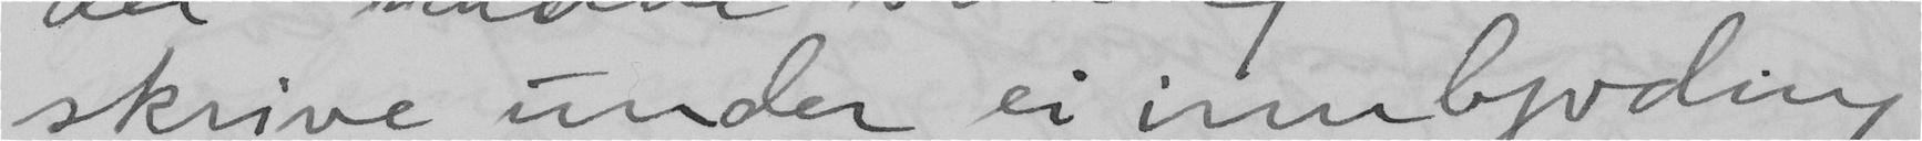skrive under ei innbjoding
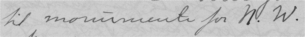til monumente for N.W.
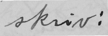skriv:
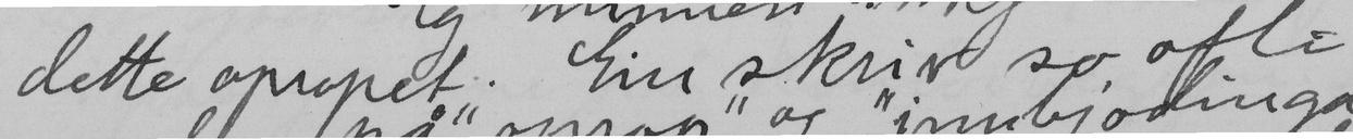dette opropet. Ein skriv so ofte
